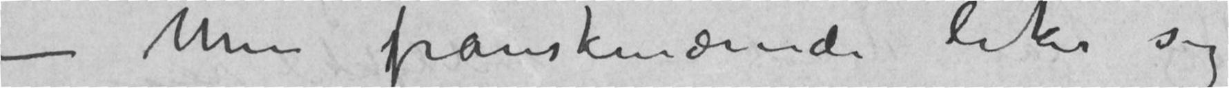– Men franskmænd liker sig
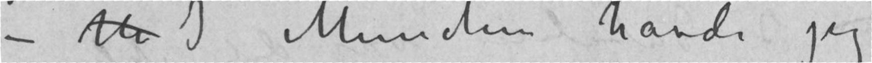–I München havde jeg
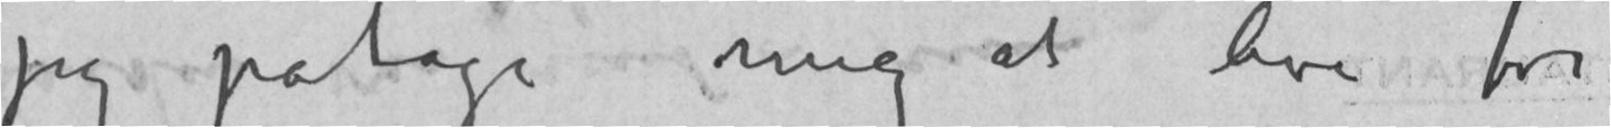jeg påtage mig at leve for
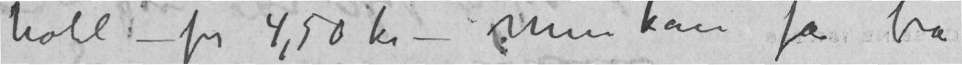hotel – for 4,50 kr – Men kan få bra
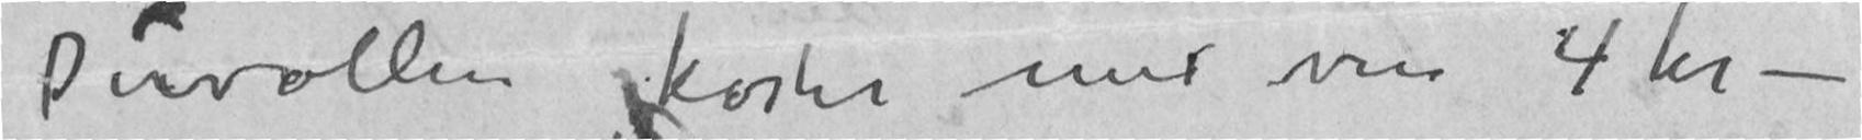koster med vin 4 kr –
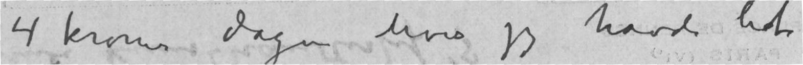4 kroner dagen hvis jeg havde lidt
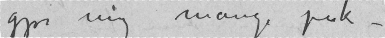gjør mig mange pek –
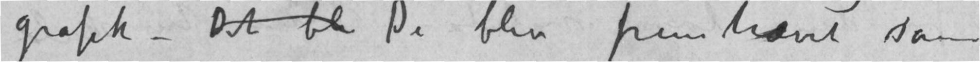grafik – Det ble De blev framhævet som
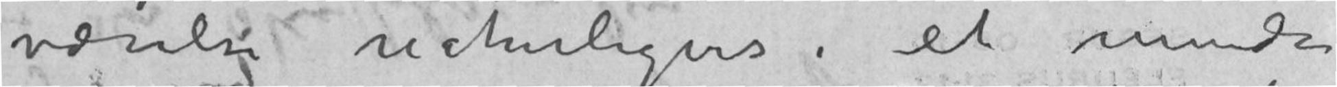værelse naturligvis i et mindre
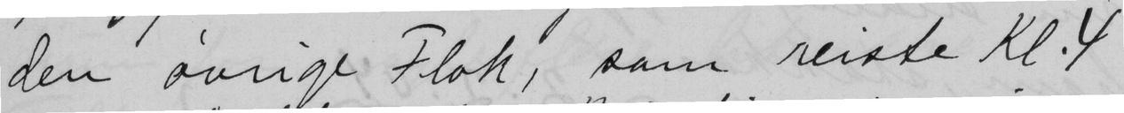den øvrige Flok, som reiste Kl. 4
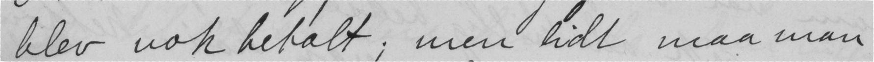blev nok betalt; men lidt maa man
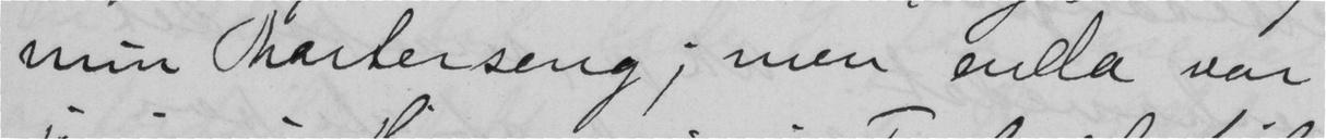min Marterseng; men enda var
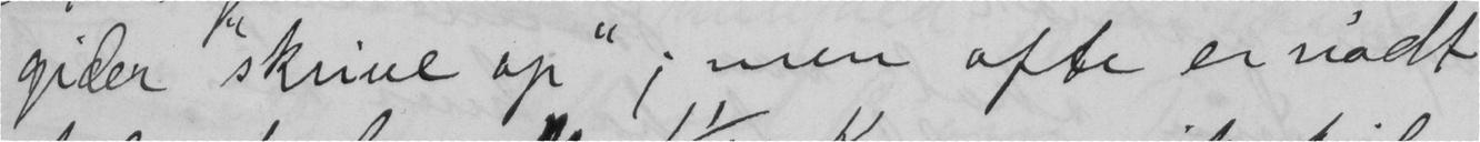gider "skrive op"; men ofte er nødt
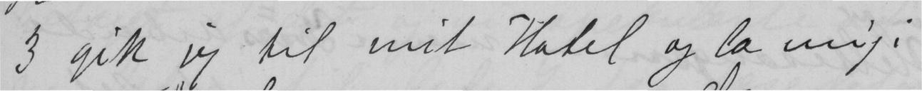3 gik jeg til mit Hotel og la mig i
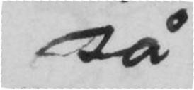så
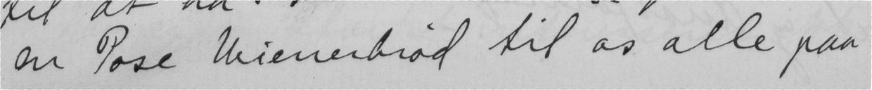en Pose Wienerbrød til os alle paa
